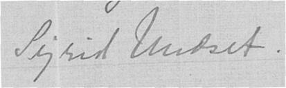Sigrid Undset.
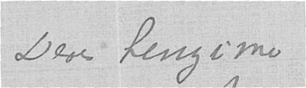Dere hengivne
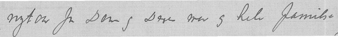nytaar for Dem og Deres mor og hele familien
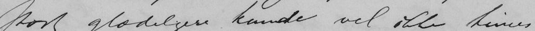stort glædeligere kunde vel ikke times
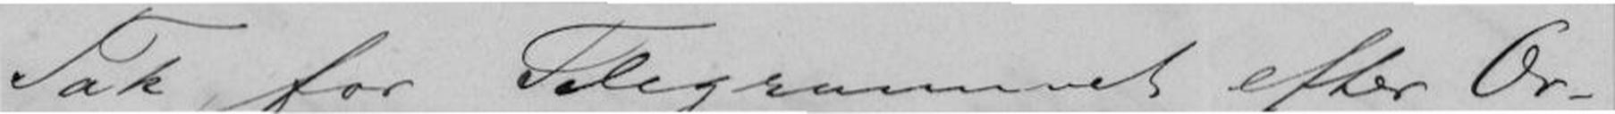Tak for Telegrammet efter Or-
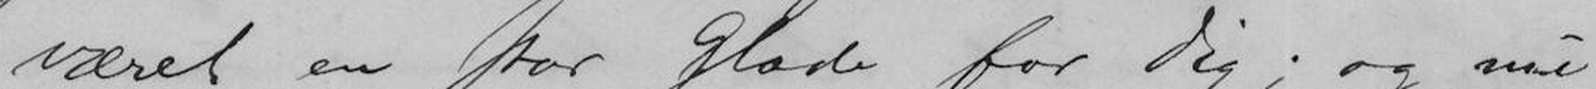været en stor Glæde for dig; og nu
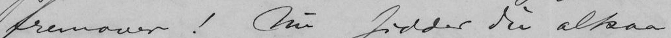fremover! Nu sidder du altsaa
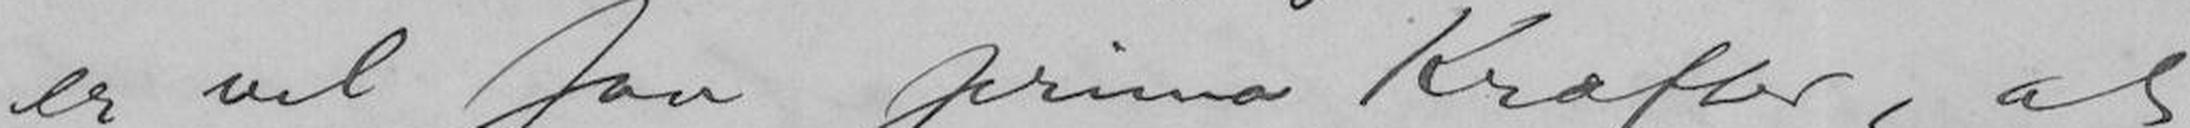er vel saa prima Kræfter, at
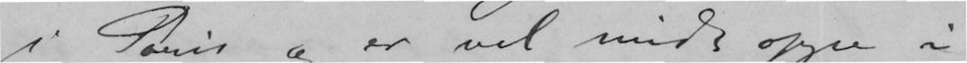i Paris og er vel midt oppe i
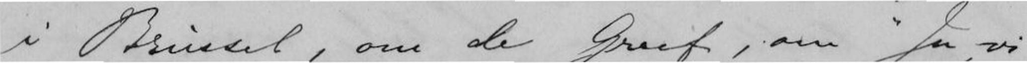i Brüssel, om de Greef, om "Ja, vi
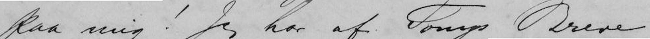paa mig! Jeg har af Tonys Breve
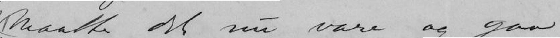dig og hende. Maatte det nu vare og gaa
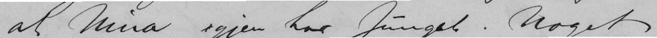at Nina igjen har sunget. Noget
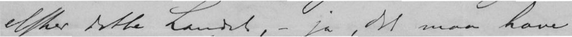elsker dette Landet, - ja, det maa have
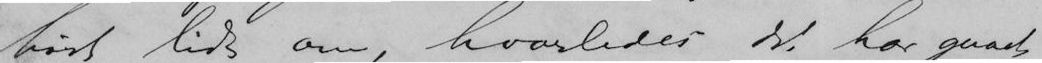hørt lidt om, hvorledes det har gaaet
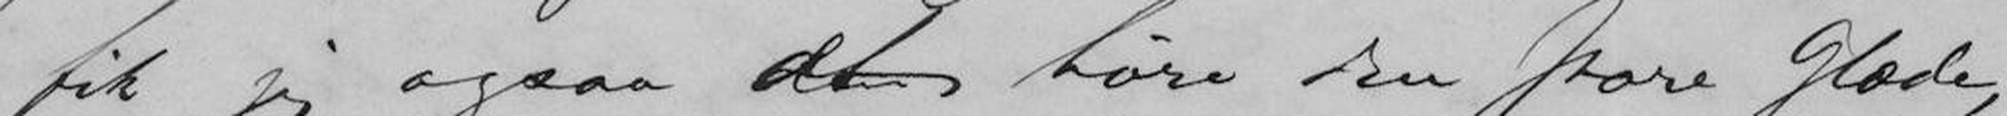fik jeg ogsaa den at høre den store Glæde,
